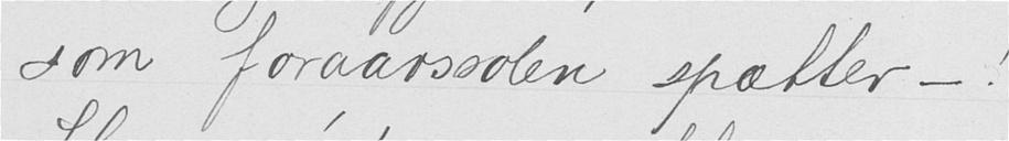som foraarssolen spætter - !
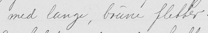med lange, brune fletter.
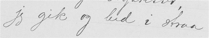jeg gik og leed i straa.
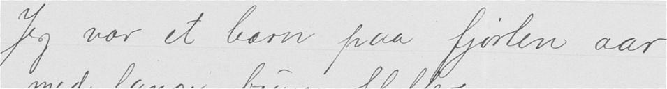Jeg var et barn paa fjorten aar
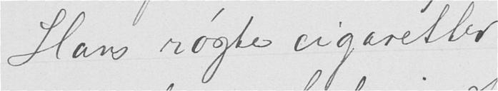Hans røgte cigaretter,
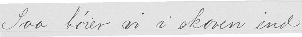Saa bøier vi i skoven ind
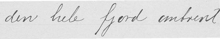den hele fjord omtrent.
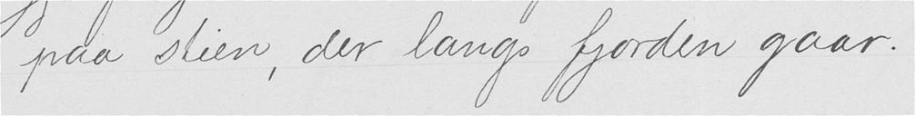paa stien, der langs fjorden gaar.
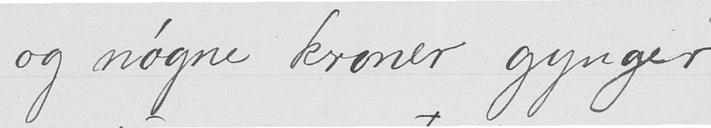og nøgne kroner gynger
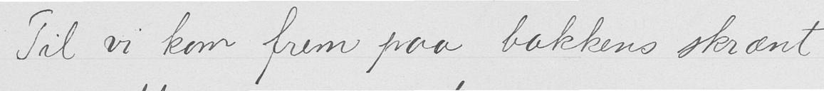Til vi kom frem paa bakkens skrænt
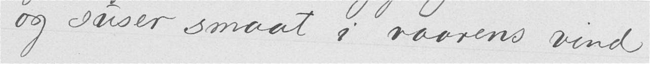og suser smaat i vaarens vind
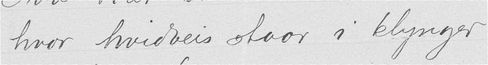hvor hvidveis staar i klynger
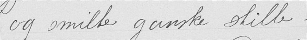og smilte ganske stille -.
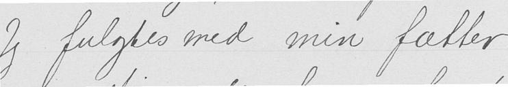Jeg fulgtes med min fætter
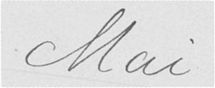Mai.
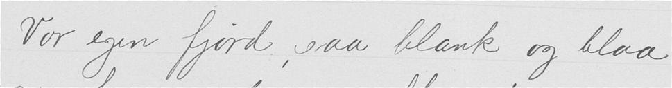- Vor egen fjord, saa blank og blaa
